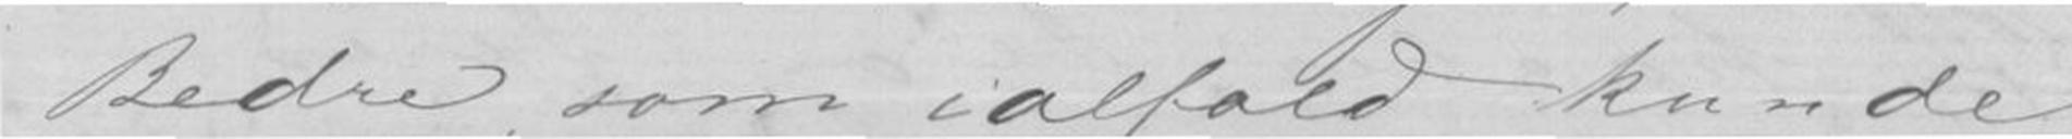Bedre, som ialfald kunde
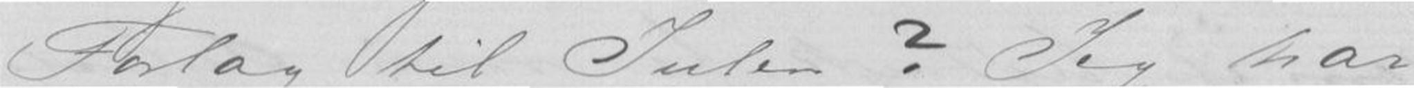Forlag til Julen? Jeg har
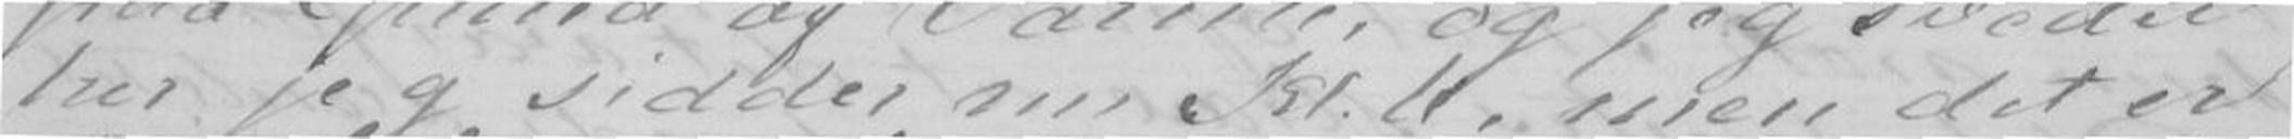her jeg sidder nu Kl.11, men det er
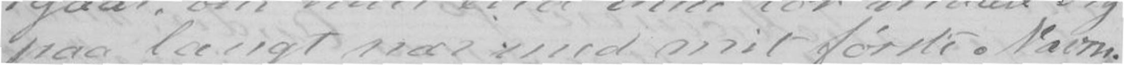paa langt nær med mit første Navn.
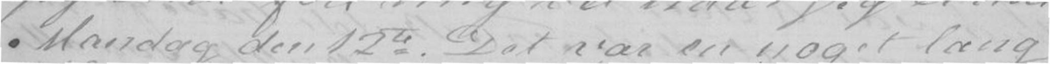Mandag den 12te. Det var en noget lang
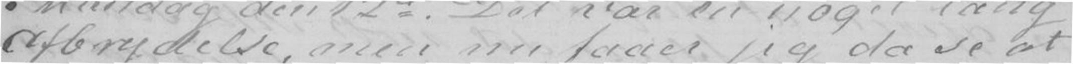Afbrytelse, men nu faaer jeg da se at
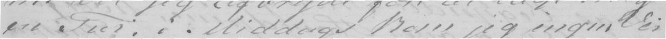en Tur, i Middags kom jeg ingen Vei
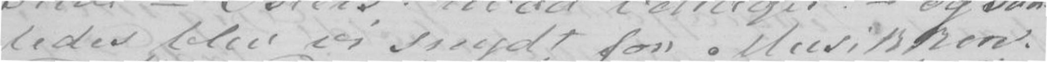ledes blev vi snydt for Musikken.
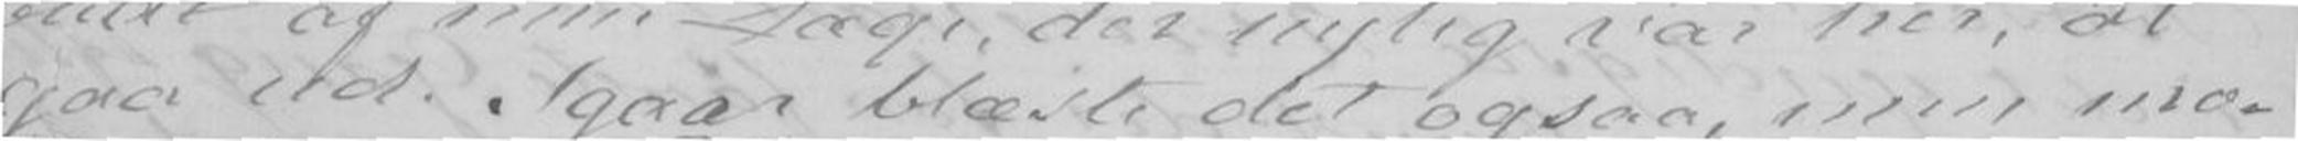gaa ud. Igaar blæste det ogsaa, men mo-
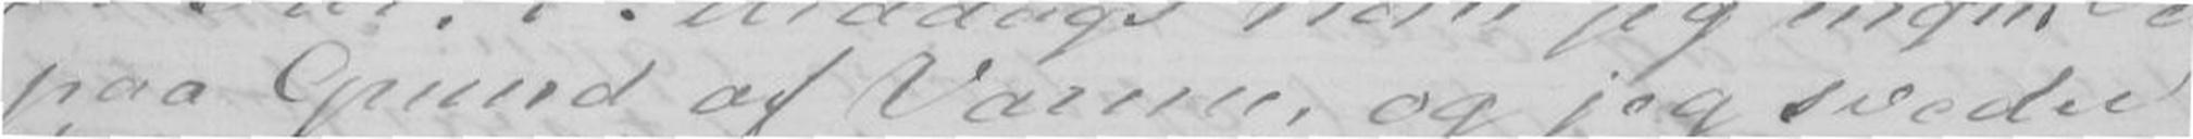paa Grund af Varme, og jeg sveder
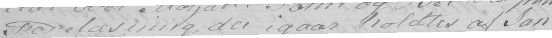Forelæsning der igaar holdes af Jan
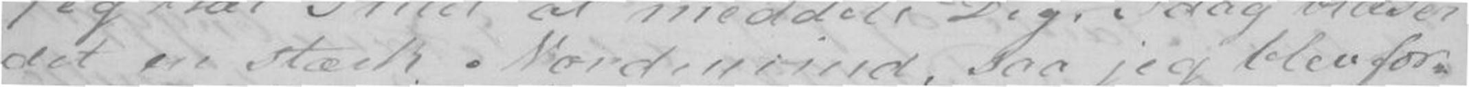det en stærk Nordenvind, saa jeg blev for-
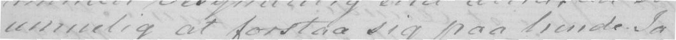umuelig at forstaa sig paa hende. Ja
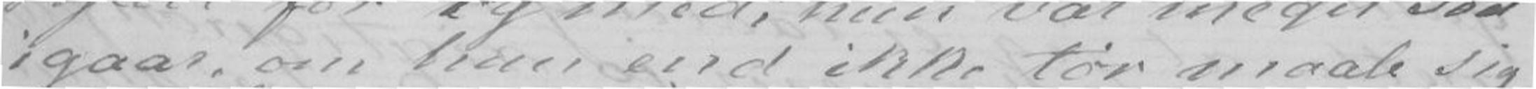igaar, om hun end ikke tør maale sig
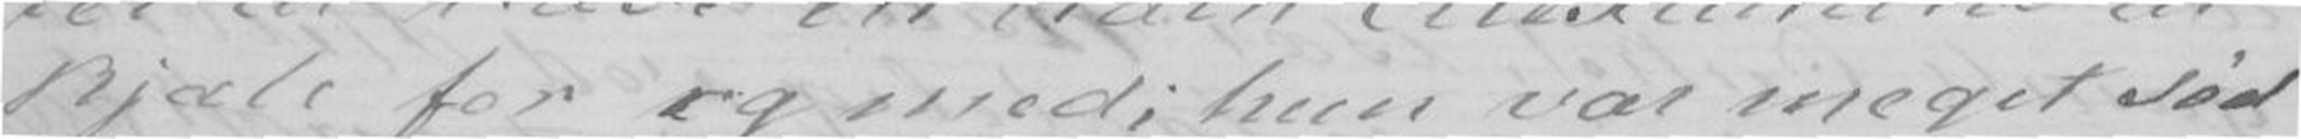kjæle for og med; hun var meget sød
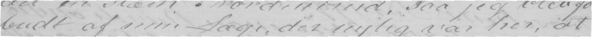budt af min Læge, der nylig var her, at
